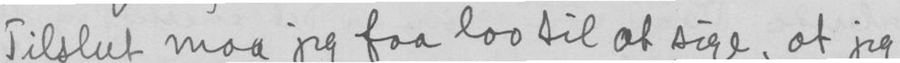Tilslut maa jeg faa lov til at sige, at jeg
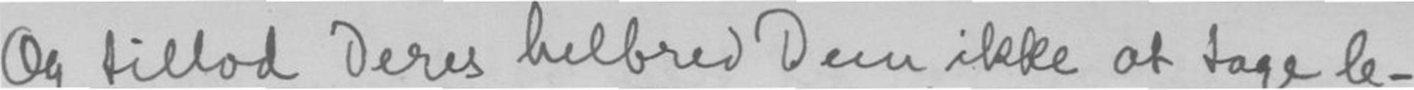Og tillod Deres helbred Dem ikke at tage le-
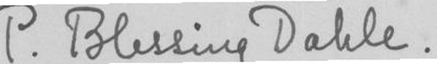P. Blissing Dahle.
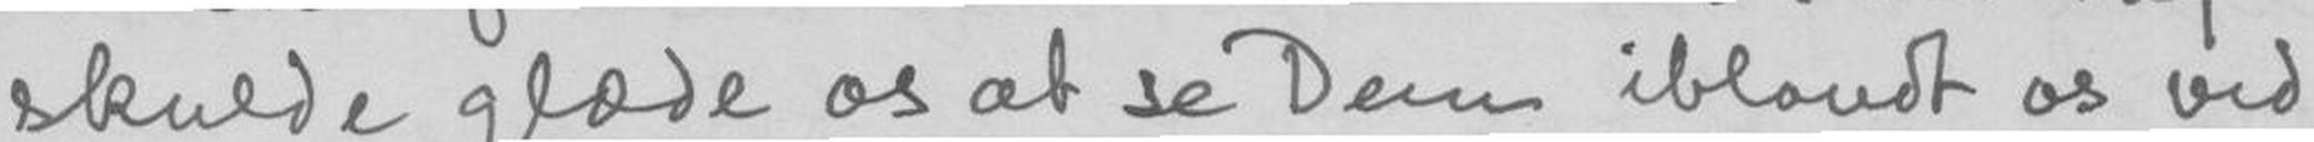skulde glæde os at se Dem iblandt os ved
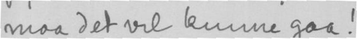maa det vel kunne gaa!
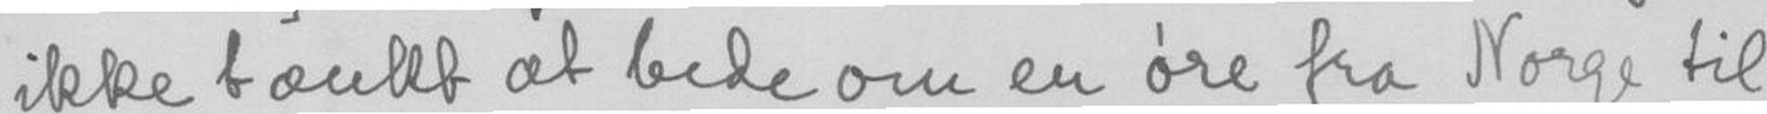ikke tænkt at bede om en øre fra Norge til
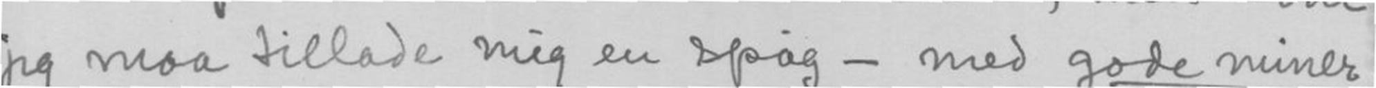jeg maa tillade mig en spøg - med gode miner
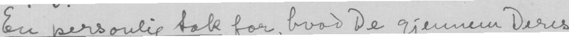En personlig tak for, hvad De gjennem Deres
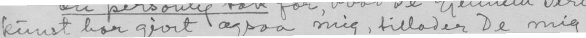kunst har gjort ogsaa mig, tillader De mig
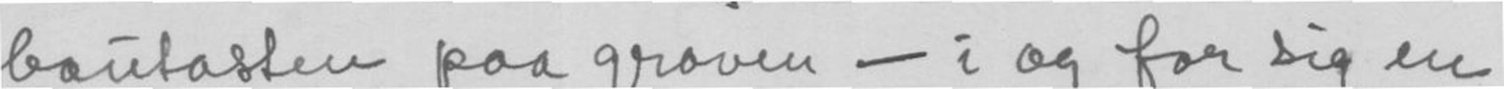bautasten paa graven - i og for sig en
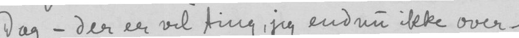Dag - der er vel ting, jeg endnu ikke over-
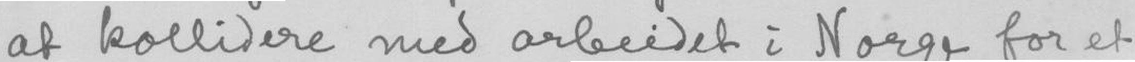at kollidere med arbeidet i Norge for et
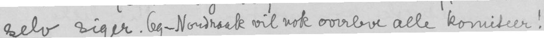selv siger. Og - Nordraak vil nok overleve alle komiteer!
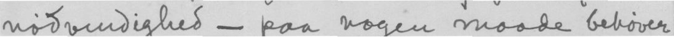nødvendighed - paa nogen maade behøver
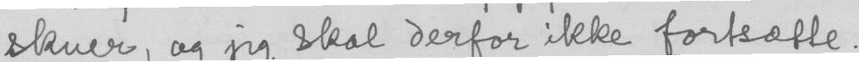skuer, og jeg skal derfor ikke fortsætte.
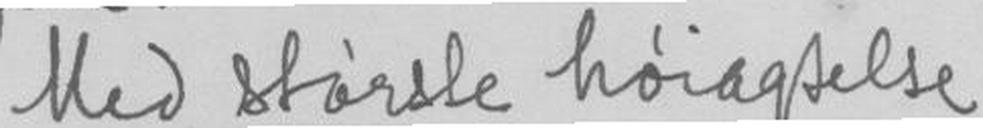Med største høiagtelse
